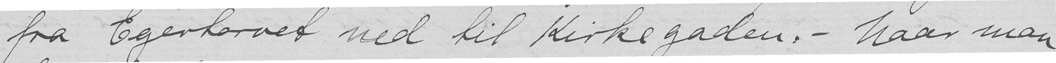fra Egertorvet ned til Kirkegaden. – Naar man
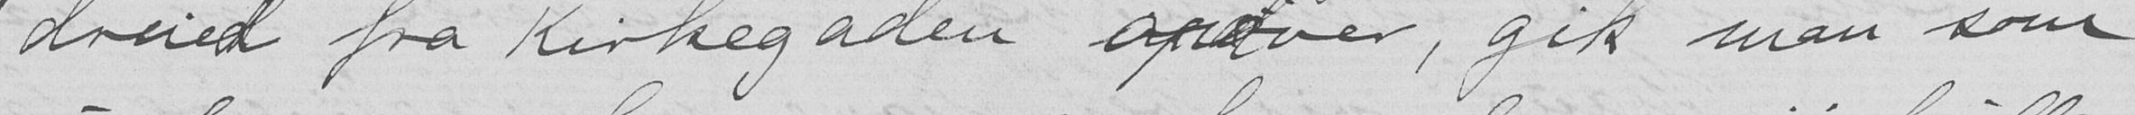dreied fra Kirkegaden opover, gik man som
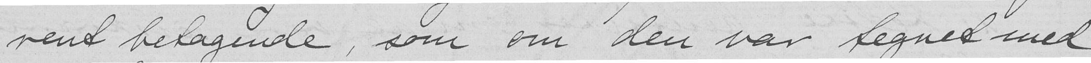rent betagende, som om den var tegnet med
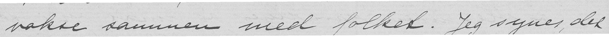vokse sammen med folket. Jeg synes, det
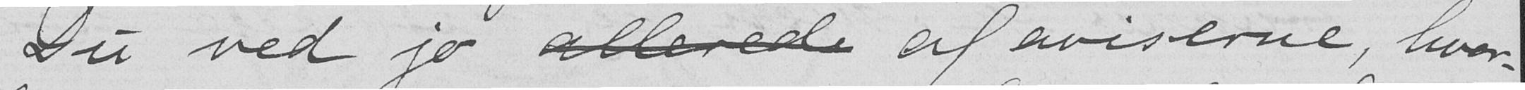Du ved jo allerede af aviserne, hvor-
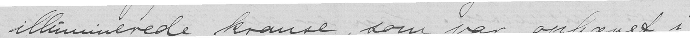illuminerede kranse, som var ophængt i
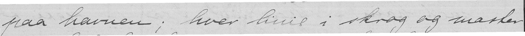paa havnen; hver linie i skrog og master
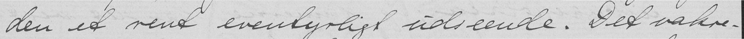den et rent eventyrligt udseende. Det vakre-
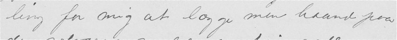ling for mig at lægge min haand paa
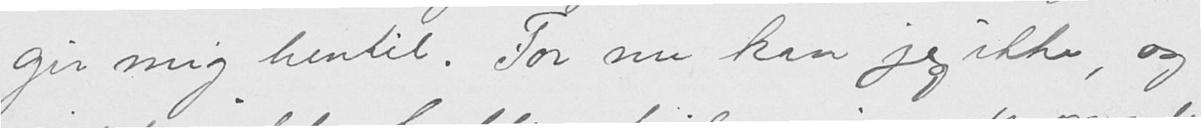gir mig hen til. For nu kan jeg ikke, og
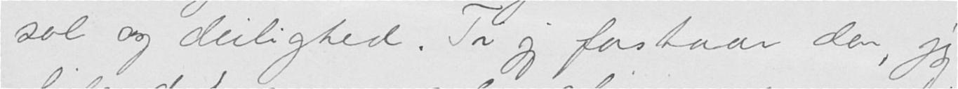sol og deilighed. Ti jeg forstaar da, jeg
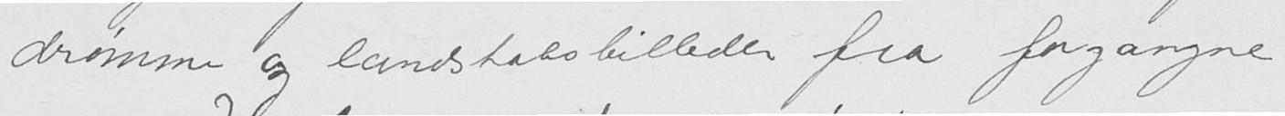drømme og landskapsbilleder fra forgangne
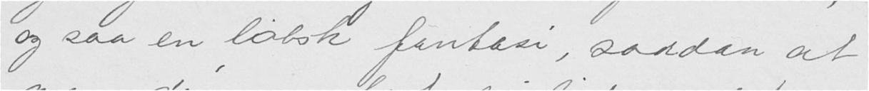og saa en løbsk fantasi, saadan at
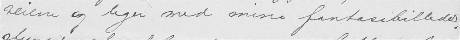veiene og leger med mine fantasibillede
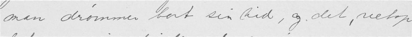man drømme bort sin tid, og det, netop
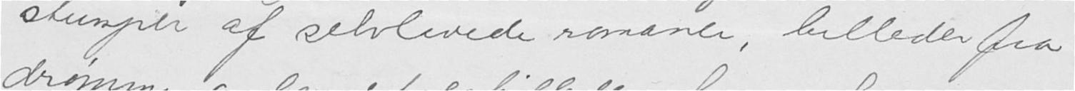stumper af selvlevede romaner, billeder fra
